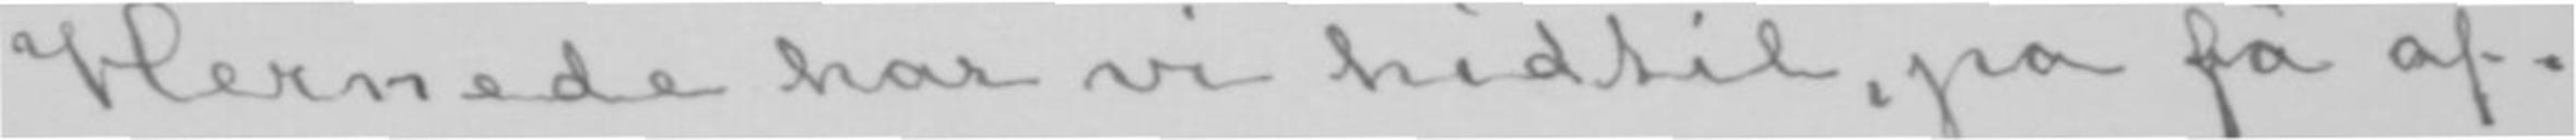Hernede har vi hidtil, på få af-
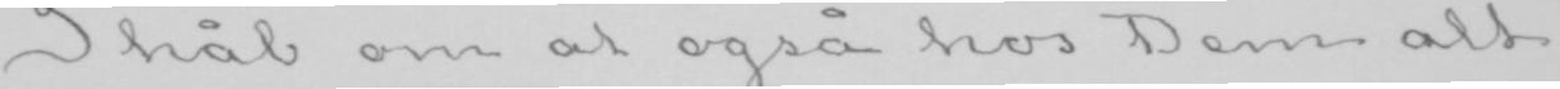I håb om at også hos Dem alt
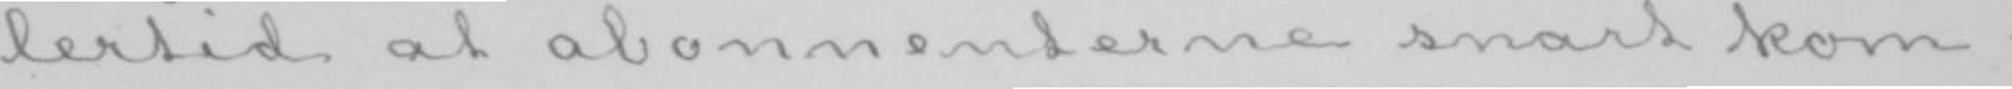lertid at abonnenterne snart kom-
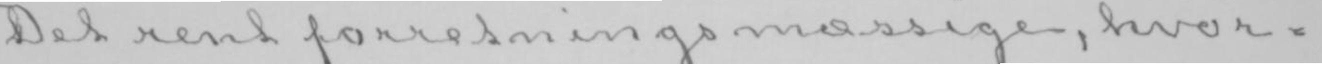Det rent forretningsmæssige, hvor-
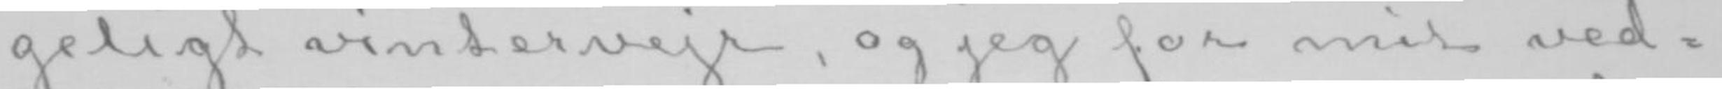geligt vintervejr, og jeg for mit ved-
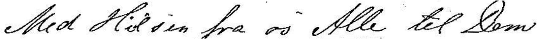Med Hilsen fra os Alle til Dem
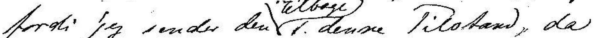fordi jeg sender den i denne Tilstand, da
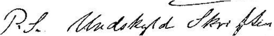P. S. Undskyld Skriften
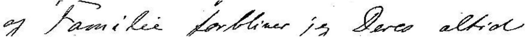og Familie forbliver jeg Deres altid
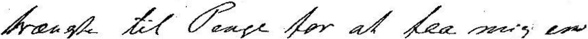trængte til Penge for at faa mig en
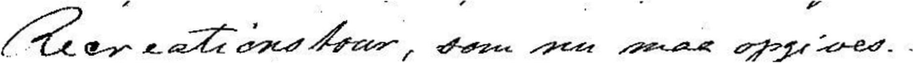Recreationstour, som nu maa opgives.-
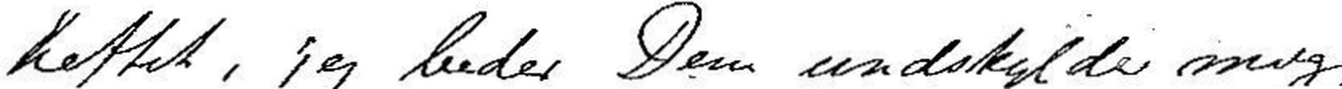heftet, jeg beder Dem undskylde mig,
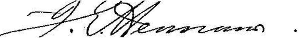J. E. Hennum.
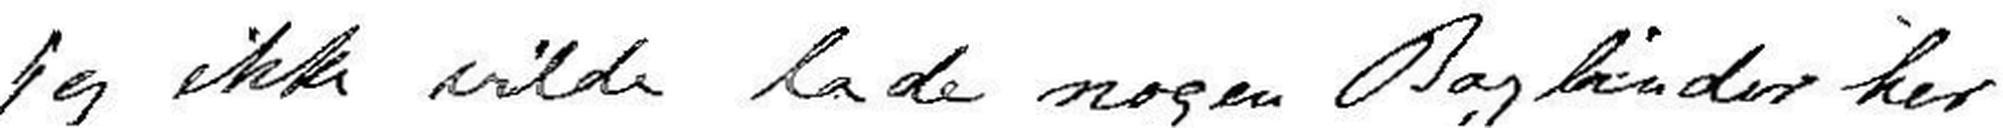jeg ikke vilde lade nogen Bogbinder her
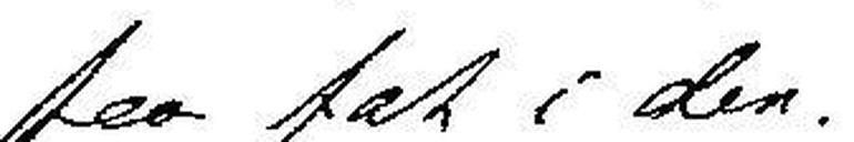faa tak i den.
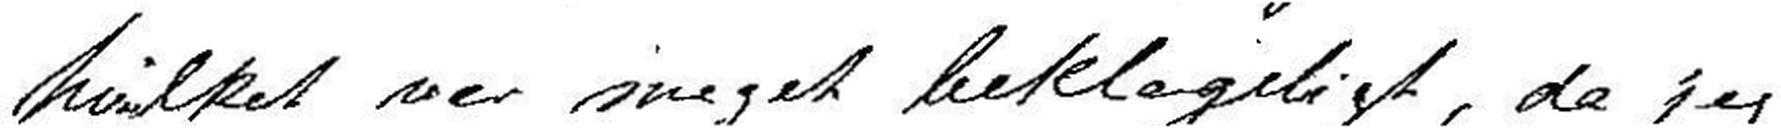hvilket var meget beklageligt, da jeg
